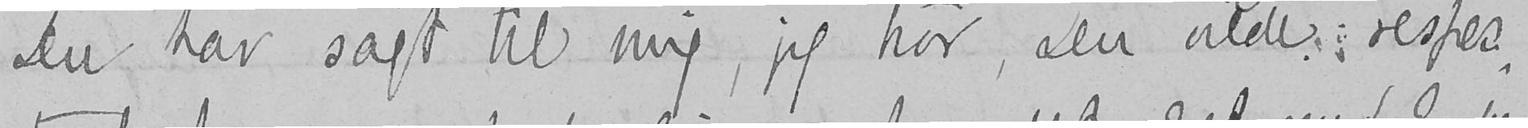Du har sagt til mig, jeg tror, Du vilde respek-
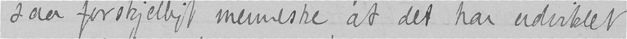saa forskjelligt menneske, at det har udviklet
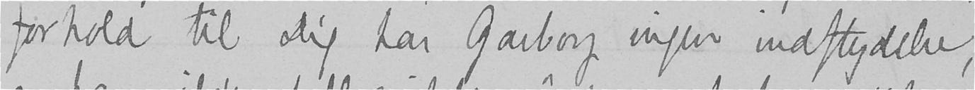forhold til Dig har Garborg ingen indflydelse,
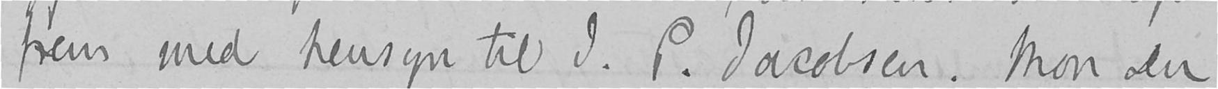frem med hensyn til J. P. Jacobsen. Mon Du
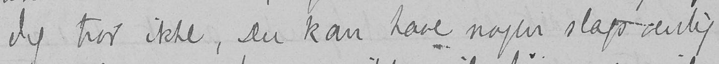Jeg tror ikke, Du kan have nogen slags venlig-
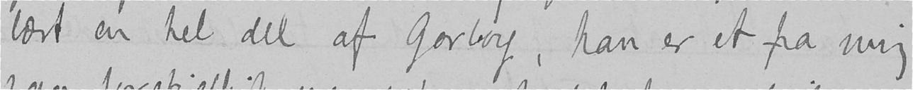lært en hel del af Garborg, han er et fra mig
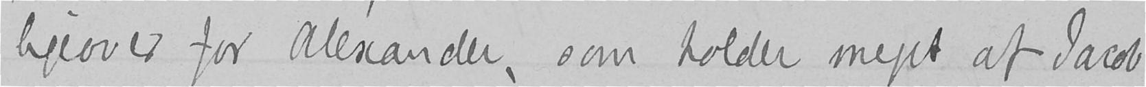ligeover for Alexander, som holder meget af Jacob-
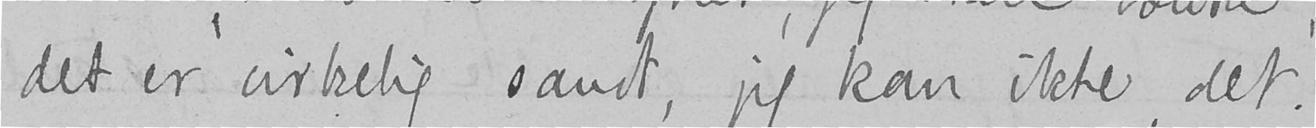det er virkelig sandt, jeg kan ikke det.
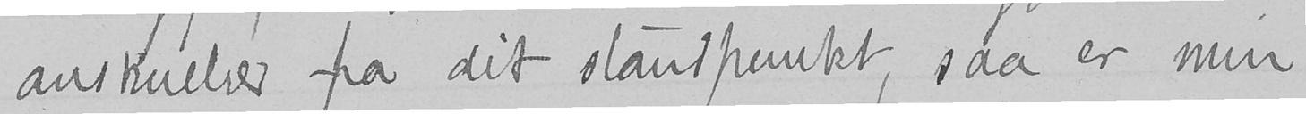anskuelse fra dit standpunkt, saa er min
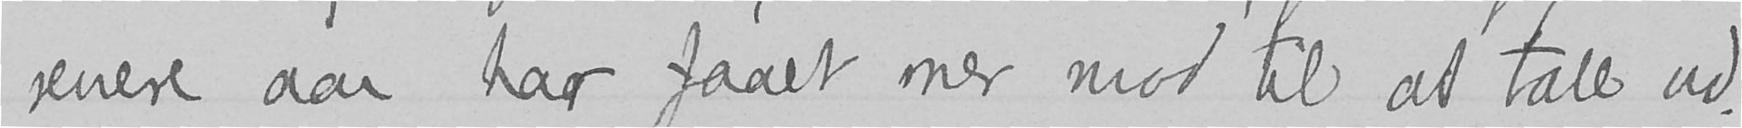senere aar har faaet mer mod til at tale ud.
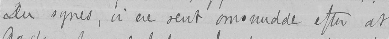Du synes, vi ere rent omsnudde efter at
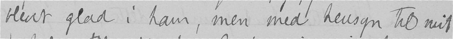blevet glad i ham, men med hensyn til mit
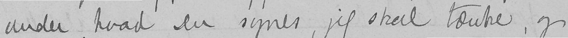under, hvad Du synes, jeg skal tænke, og
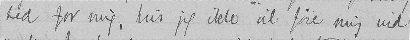hed for mig, hvis jeg ikke vil føre mig ind
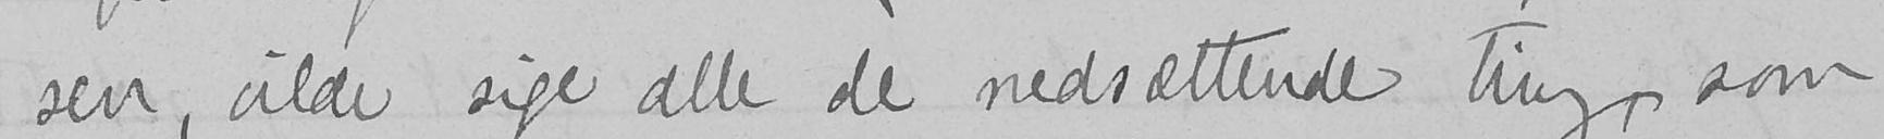sen, vilde sige alle de nedsættende ting, som
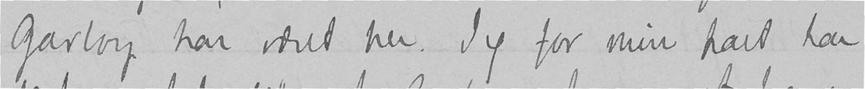Garborg har været her. Jeg for min part har
